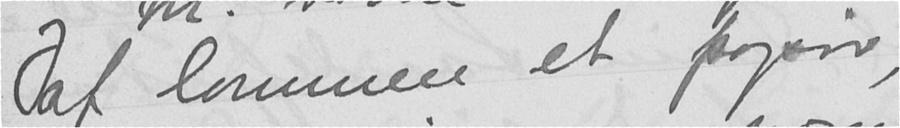af lommen et papir,
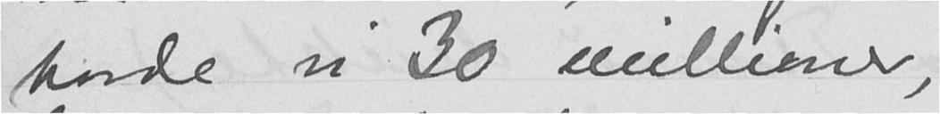havde vi 30 millioner,
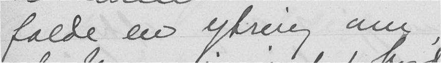falde en ytring om,
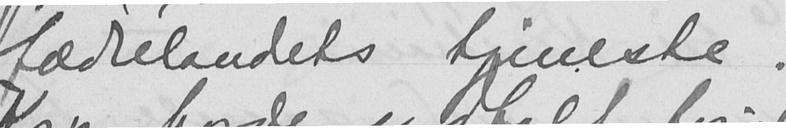fædrelandets tjeneste.
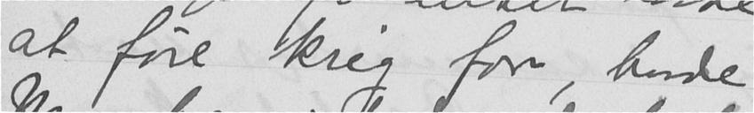at føre krig for, havde
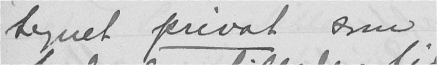tegnet privat som
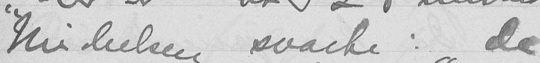Michelsen svarte: "de
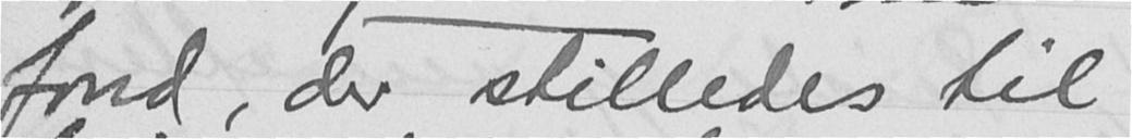fond, der stilledes til
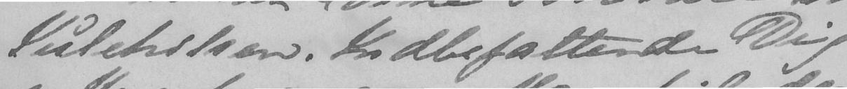Julehilsen. Indbefattende Dig
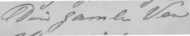Din gamle Ven
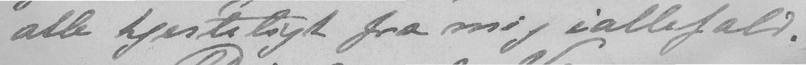alle hjerteligt fra mig iallefald.
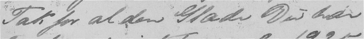Tak for al den Glæde Du har
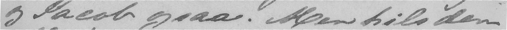og Jacob ogsaa. Men hils dem
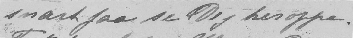snart faa se Dig heroppe.
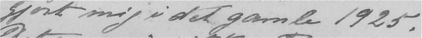gjort mig i det gamle 1925.
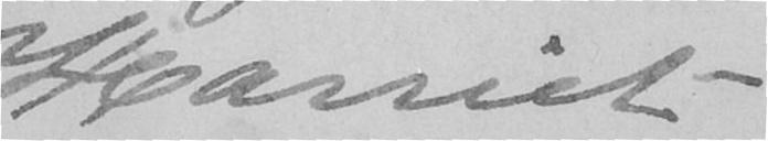Harriet
